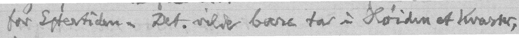for Eftertiden. Det vilde bare ta i Høiden et Kvarter,
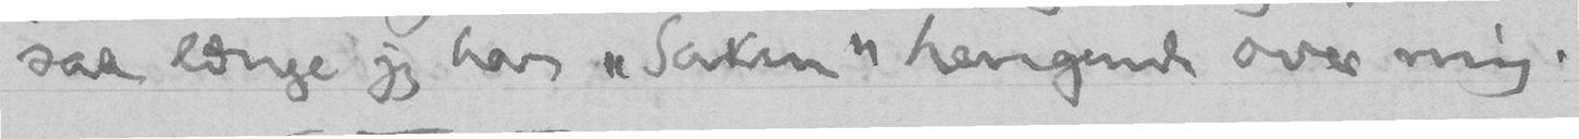saa længe jeg har "Saken" hengende over mig.
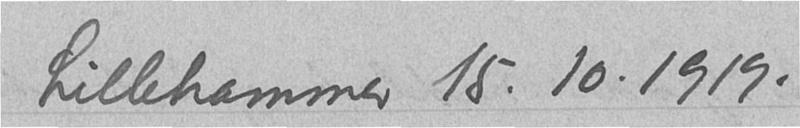Lillehammer 15.10.1919.
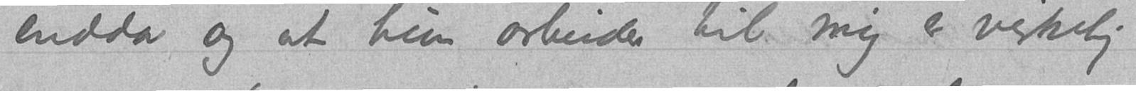endda og at hun arbeider til mig er virkelig
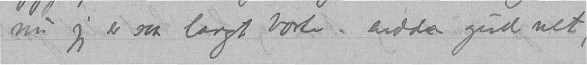nu jeg er saa langt borte – endda gud vet,
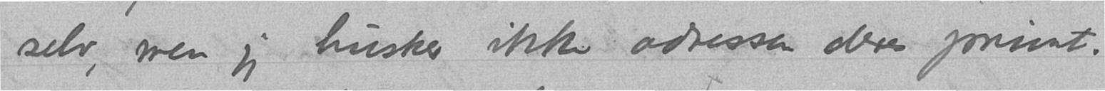selv, men jeg husker ikke adressen deres privat.
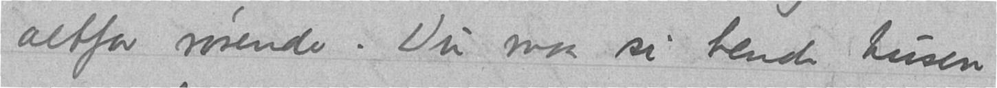altfor rørende. Du maa si hende tusen
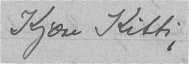Kjære Kitti,
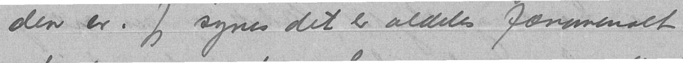den er. Jeg synes det er aldeles fænomenalt
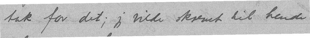tak for det; jeg vilde skrevet til hende
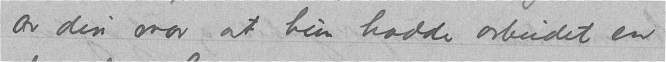av din mor at hun hadde arbeidet en
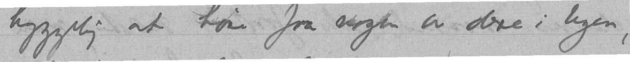hyggelig at høre fra nogen av dere i byen,
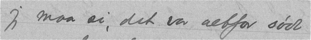jeg maa si, det var altfor sødt
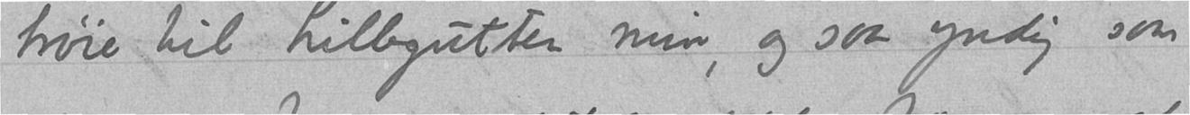trøie til Lillegutten min, og saa yndig som
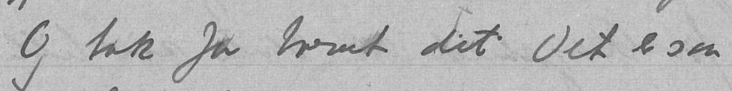Og tak for brevet dit. Det er saa
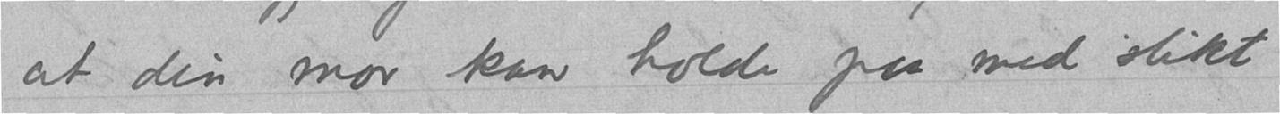at din mor kan holde paa med slikt
